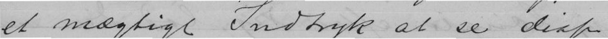et mægtigt Indtryk at se disse
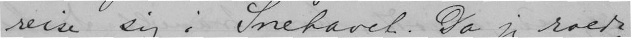reise sig i Snehavet. Da jeg roede
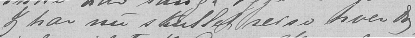Jeg har nu skullet reise hver dag
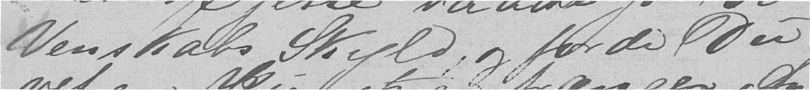Venskabs Skyld, og fordi Du
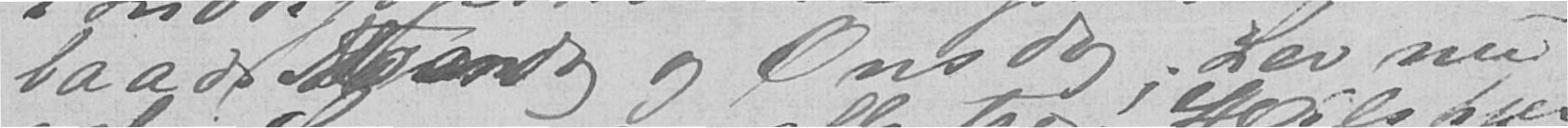baade Mandag og Onsdag. Lev nu
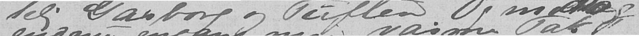telig Garborg og Tuften. Og modtag
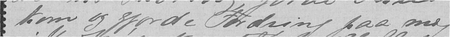kom og gjorde Fordring paa mig
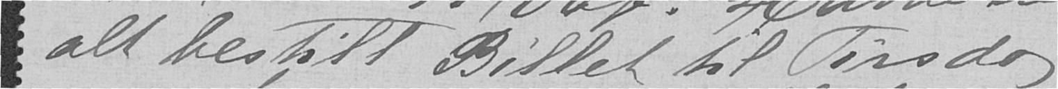alt bestilt Billet til Tirsdag,
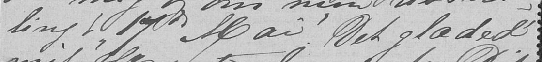ling i 17de Mai! Det glæded'
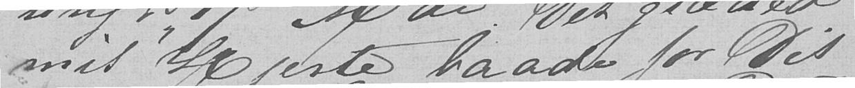mit Hjerte baade for Dit
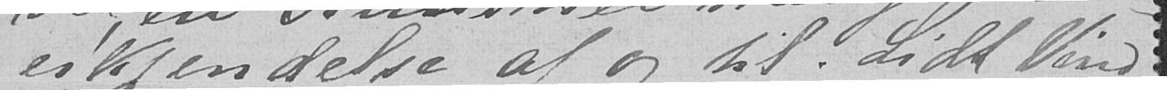erkjendelse af og til! Lidt Vind
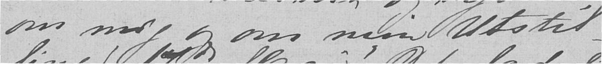om mig og om min Utstil-
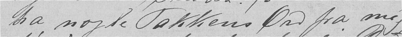ha nogle Takkens Ord fra mig
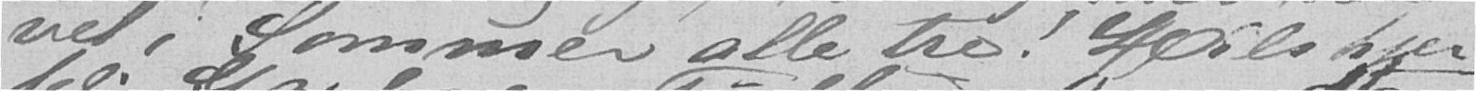vel i Sommer alle tre! Hils hjer
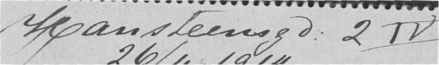Hansteensgd. 2 IV
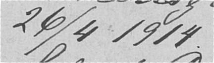26/4 1914
